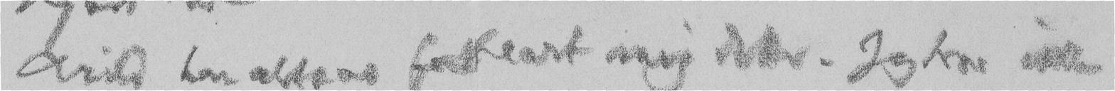Arild har altsaa forklart mig dette. Jeg tror ikke
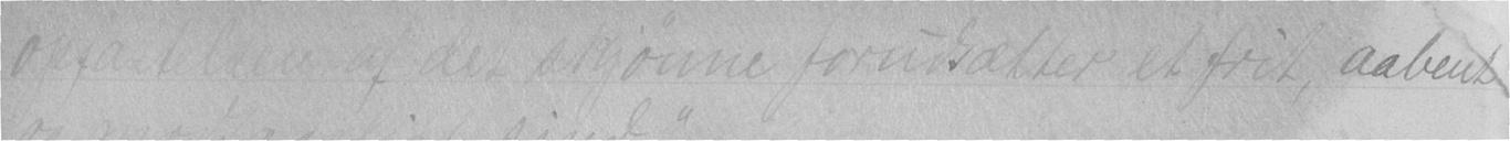opfaltelse af det skjønne forudsætter et frit, aabent
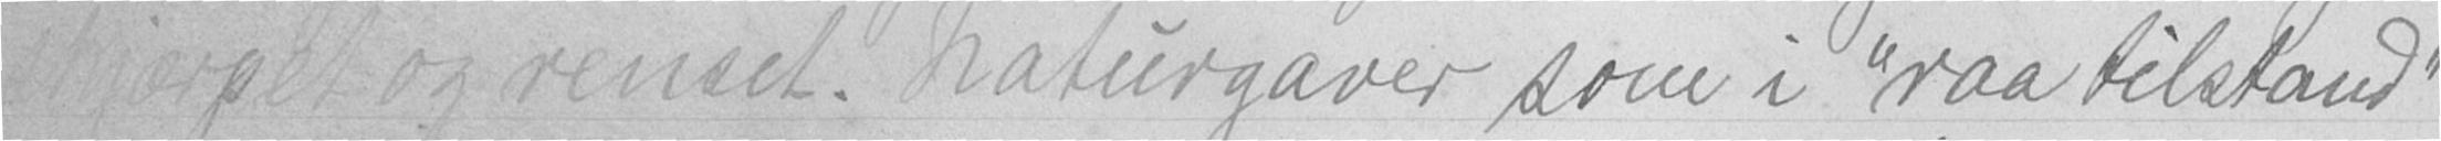skjærpet og renset. Naturgaver som i "raa tilstand"
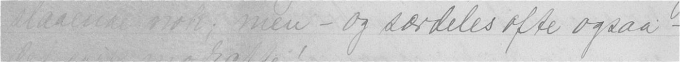slaaende nok; men - og særdeles ofte ogsaa -
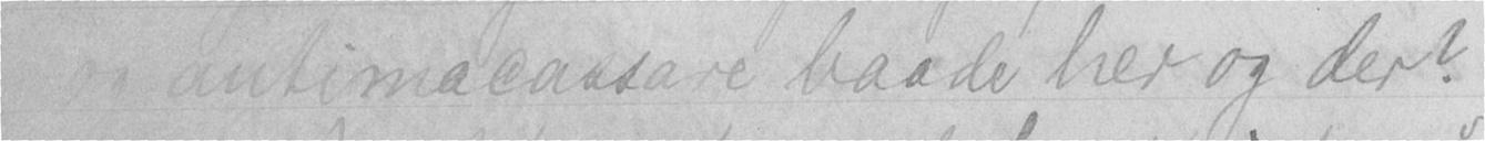og antimacassare baade her og der?
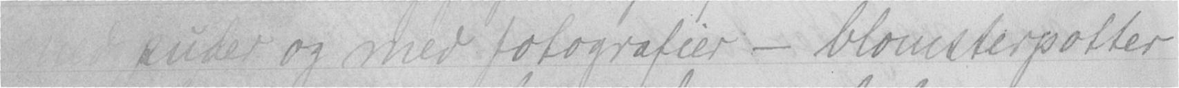, puder og med fotografier - blomsterpotter
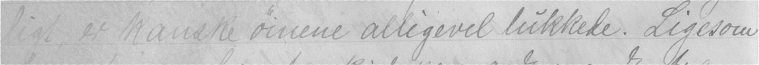ligt, er kanske øiene alligevel lukkede. Ligesom
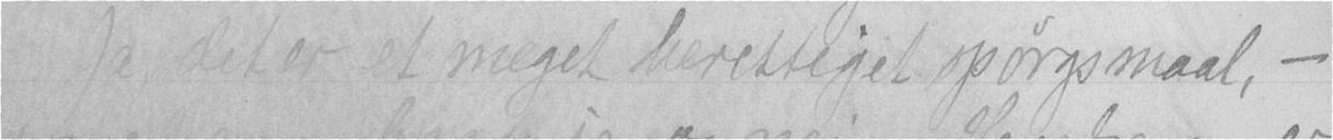Ja, det er et meget berettiget spørgsmaal, -
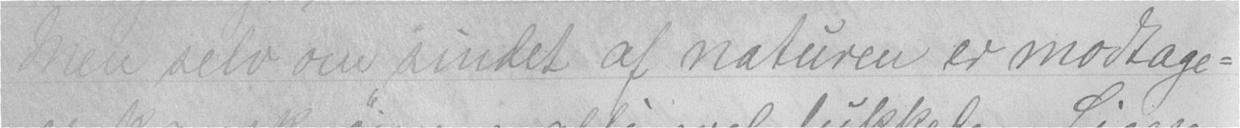Men selv om sindet af naturen er modtage-
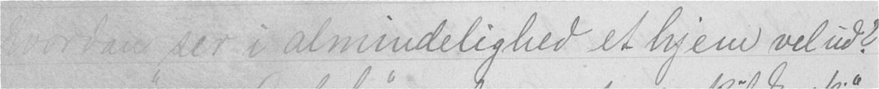Hvordan ser i almindelighed et hjem vel ud?
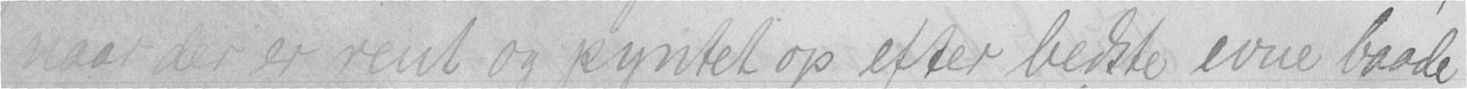naar der er rent og pyntet op efter bedste evne baade
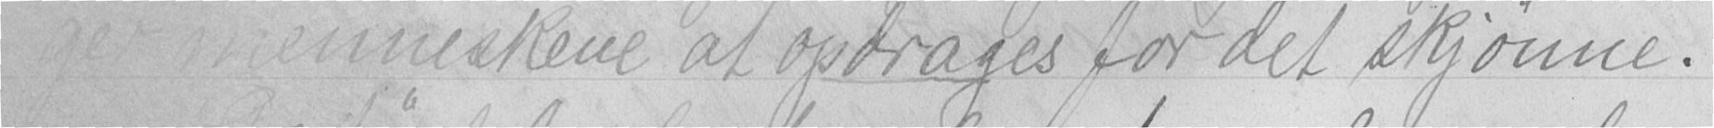ger menneskene at opdrages for det skjønne.
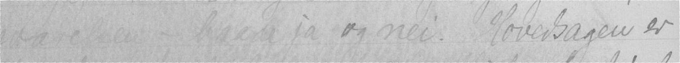svaret - baade ja og nei. Hovedsagen er
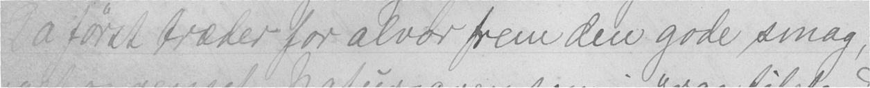Da først træder for alvor frem den gode smag,
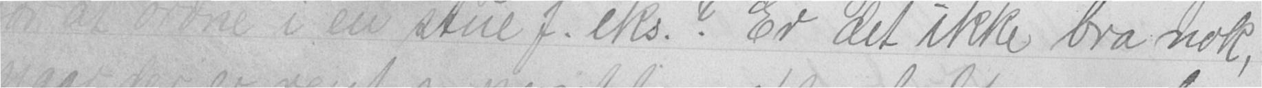for at ordne i en stue f. eks.? Er det ikke bra nok,
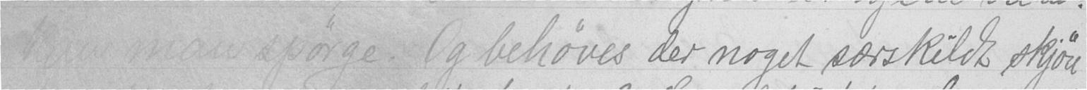kan man spørge. Og behøves der noget særskildt skjøn
